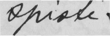spiste
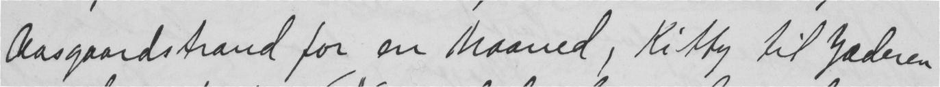Aasgaardstrand for en Maaned, Kitty til Jæderen
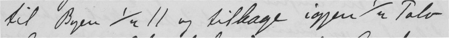til Byen 1/2 11 og tilbage igjen 1/2 Tolv
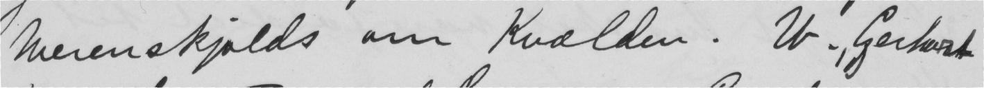Werenskjolds om Kvælden. W. Gerhart
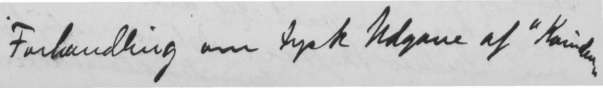Forhandling om tysk Udgave af "Kvinden"
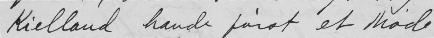Kielland havde først et Møde
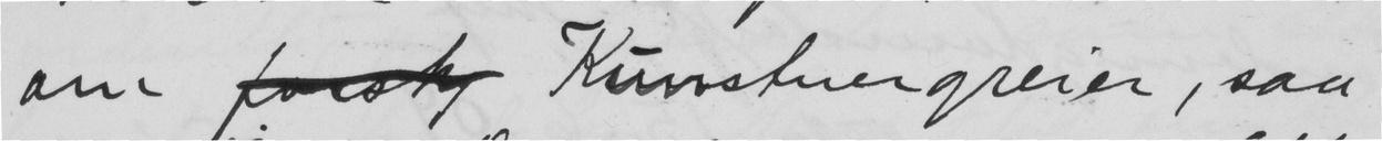om  Kunstnergreier, saa
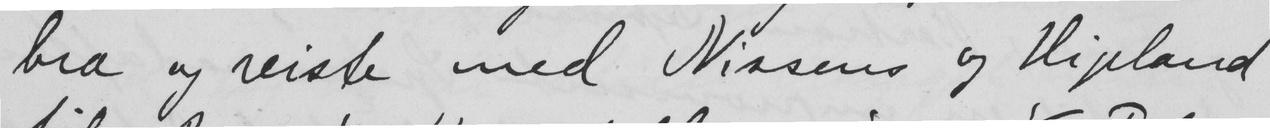bra og reiste med Nissens og Vigeland
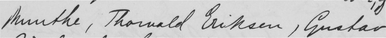Munthe, Thorvald Eriksen, Gustav
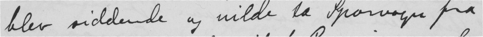blev siddende og vilde ta Sporvogn fra
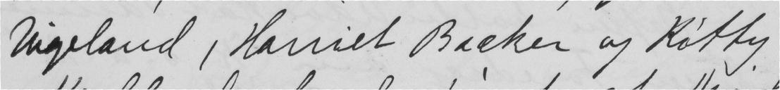Vigeland, Harriet Backer og Kitty
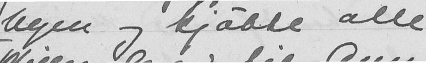byen og kjøbte alle
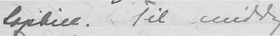Sophie. Til middag
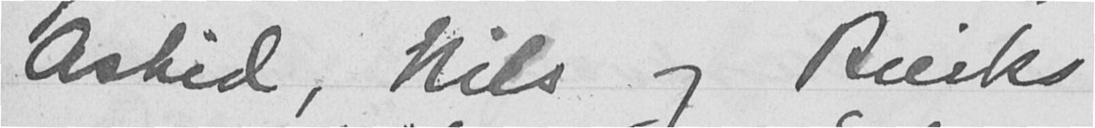Astrid, Nils og Riecks
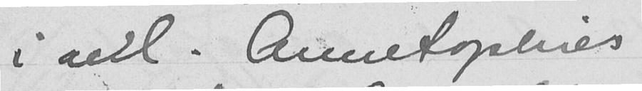i anl. Anne Sophies
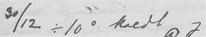30/12 ÷ 10º koldt og
